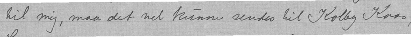til mig, maa det vel kunne sendes til Kolby Kaas,
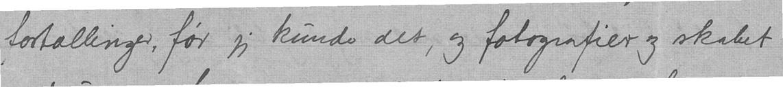fortællinger, før jeg kunde det, og fotografier og skabet
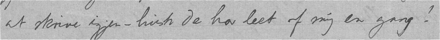at skrive igjen - husk De har leet af mig en gang!
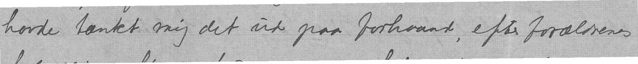havde tænkt mig det ud paa forhaand, efter forældrenes
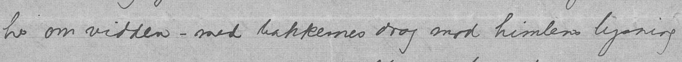her om vidden - med bakkemes drag mod himlens lysning
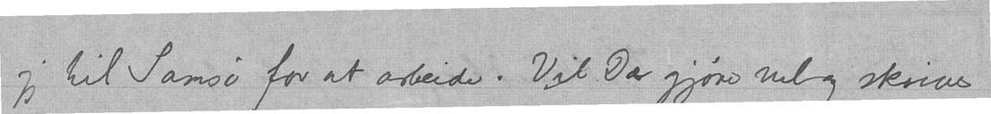jeg til Samsø for at arbeide. Vil De gjøre vel og skrive
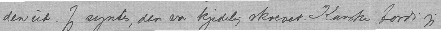den ud. Jeg syntes, den var kjedelig skrevet. Kanske fordi jeg
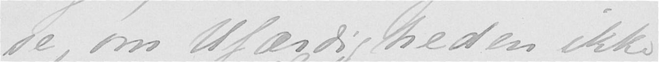se, om Ufærdigheden ikke
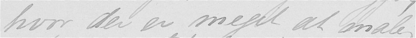hvor der er meget at male,
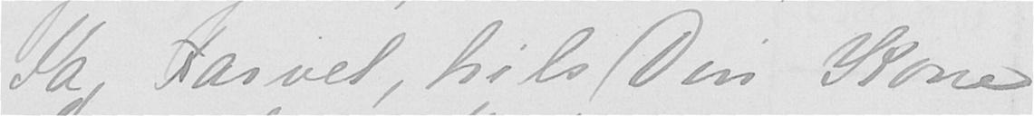Ja, Farvel, hils Din Kone
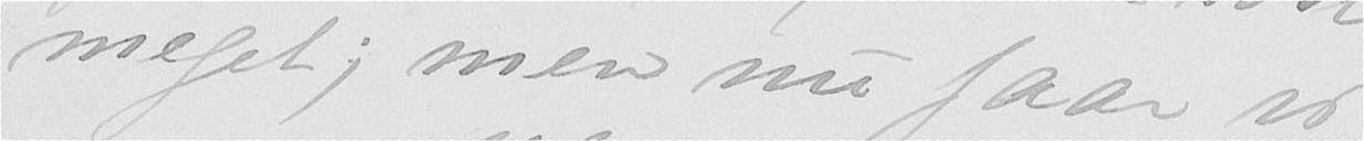meget; men nu faar vi
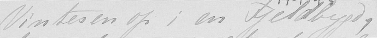Vinteren op i en Fjeldbygd,
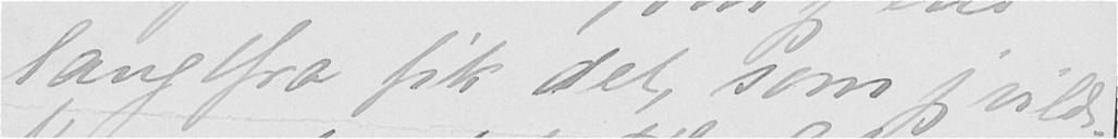langtfra fik det, som jeg vilde.
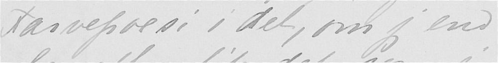Farvepoesi i det, om jeg end
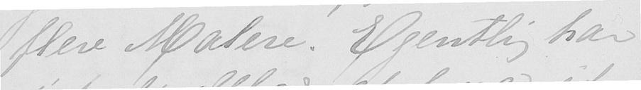flere Malere. Egentlig har
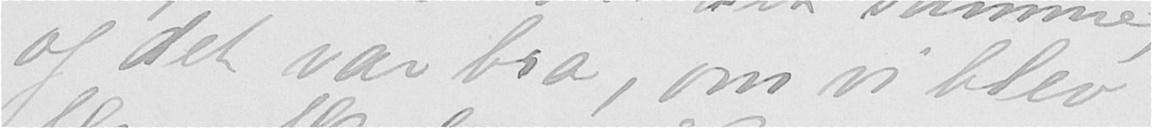og det var bra, om vi blev
